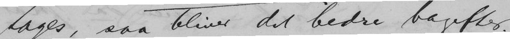tages, saa bliver det bedre bagefter.
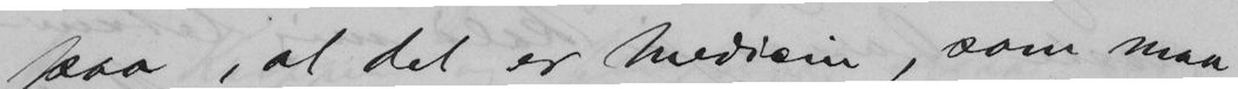paa, at det er Medicin, som maa
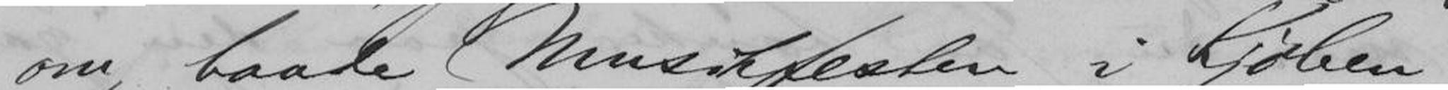om baade Musikfesten i Kjøben-
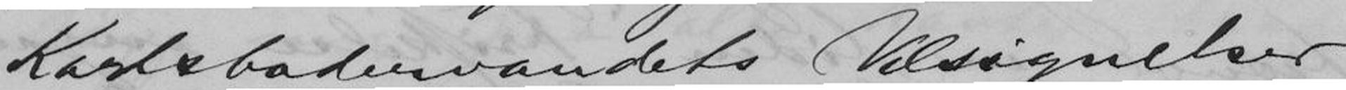Karlsbadervandets Velsignelser -
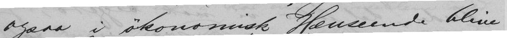ogsaa i økonomisk Henseende blive
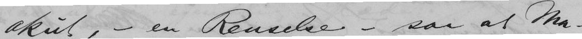akut, - en Renselse - saa at Ma-
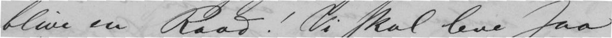blive en Raad! Vi skal leve saa
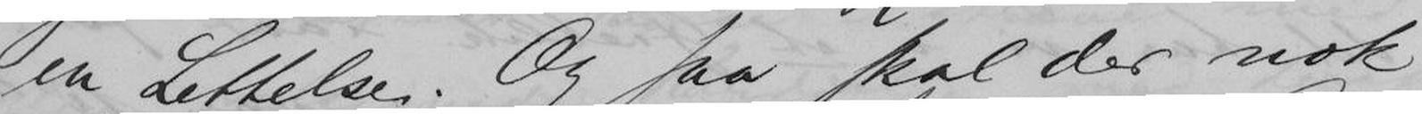en Lettelse. Og saa skal der nok
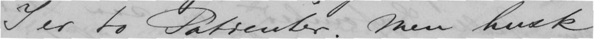I er to Patienter. Men husk
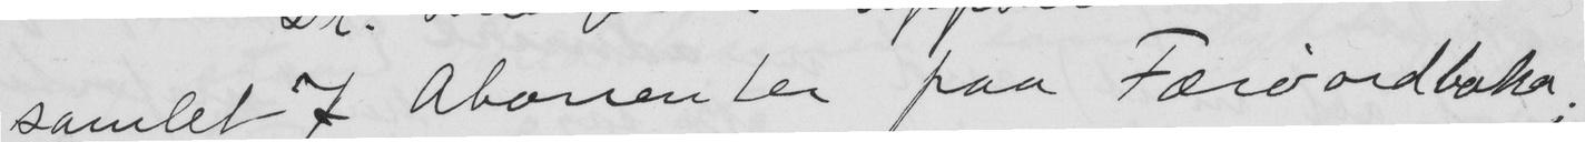samlet 7 Abonenter paa Færøordboka;
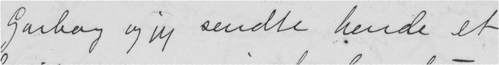Garbog og jeg sendte hende et
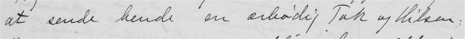at sende hende en ærbødig Tak og Hilsen.
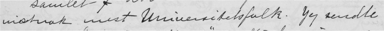vistnok mest Universitetsfolk. Jeg sendte
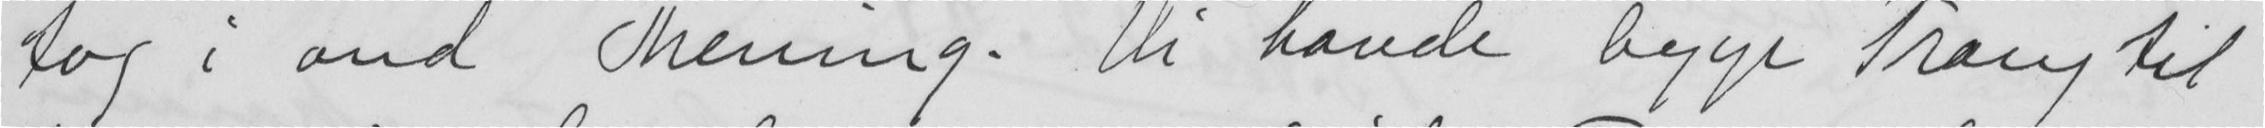tog i ond Mening. Vi havde begge Trang til
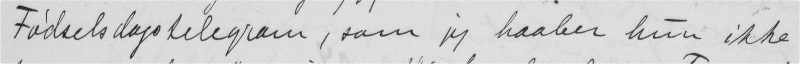Fødselsdagstelegram, som jeg haaber hun ikke
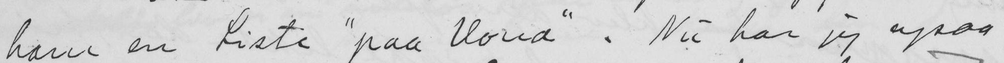ham en Liste "paa Vova". Nu har jeg ogsaa
